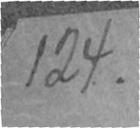124.
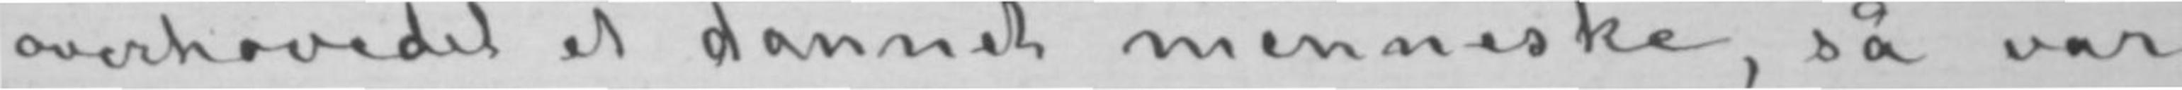overhovedet et dannet menneske, så var
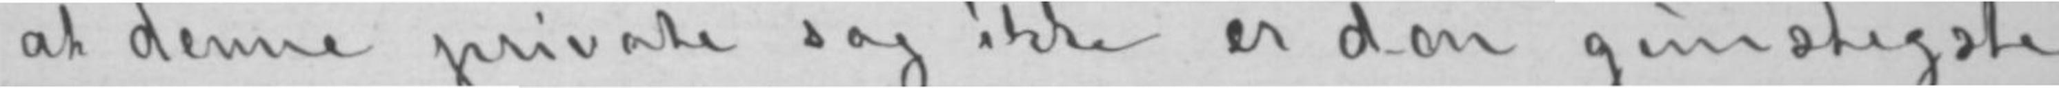at denne private sag ikke er den gunstigste
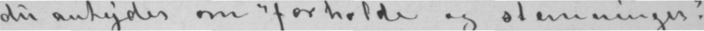du antyder om «forholde og stemninger».
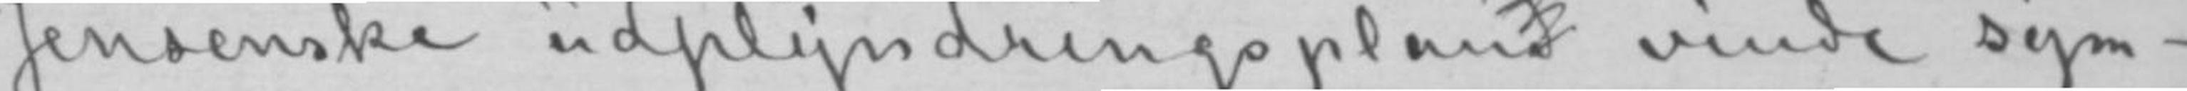Jensenske udplyndringspland vinde sym-
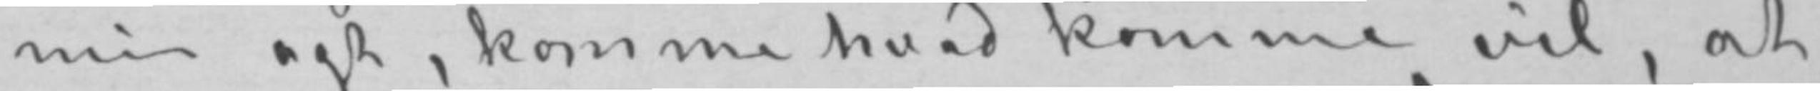min agt, komme hvad komme vil, at
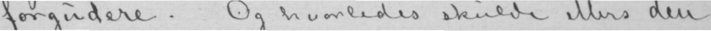forgudere. Og hvorledes skulde ellers den
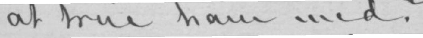at true ham med?
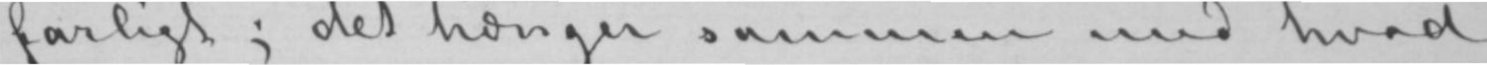farligt; det hænger sammen med hvad
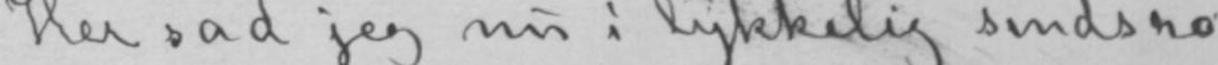Her sad jeg nu i lykkelig sindsro
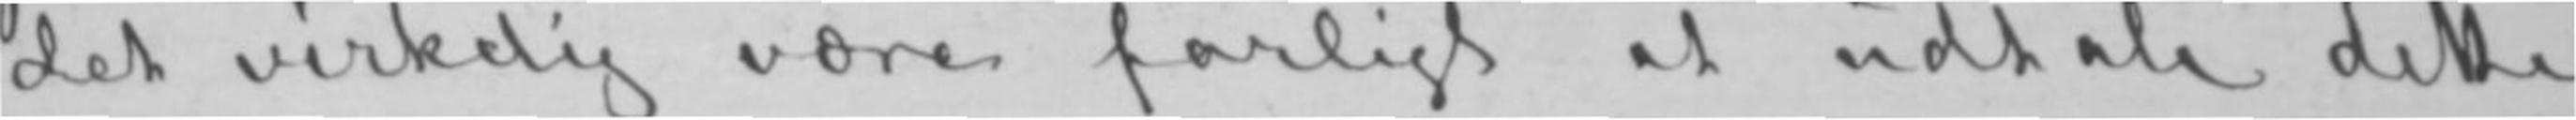det virkelig være farligt at udtale dette
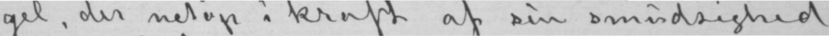gel, der netop i kraft af sin smudsighed
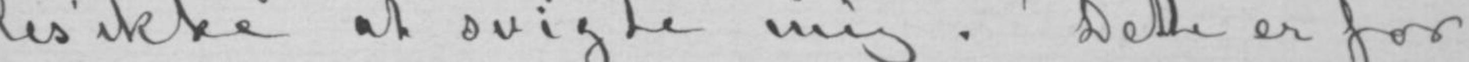les ikke at svigte mig. Dette er for
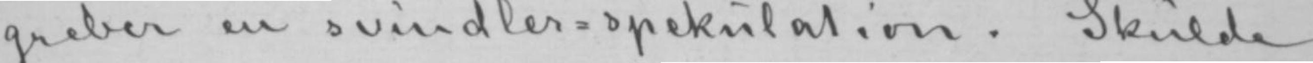greber en svindler-spekulation. Skulde
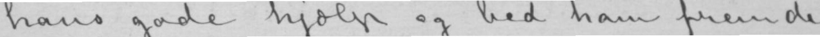hans gode hjælp og bed ham fremde-
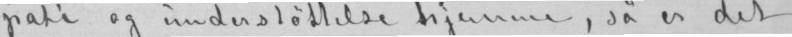pati og understøttelse hjemme, så er det
